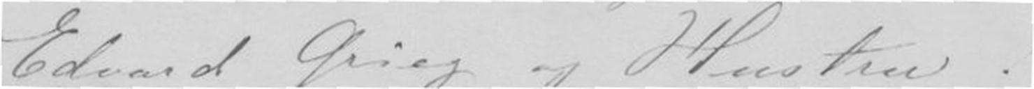Edvard Grieg og Hustru!
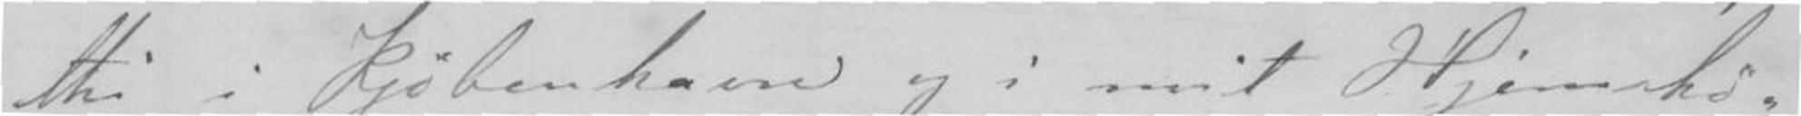thi i Kjøbenhavn og i mit Hjem?-
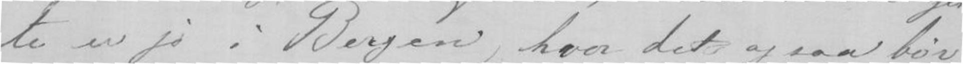te er jo i Bergen, hvor det ogsaa bør
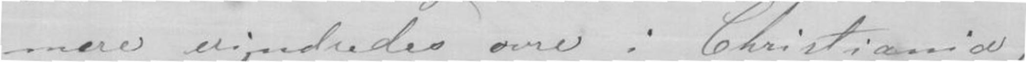mere erindredes over i Christiania,
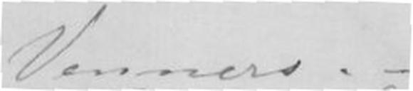Venners.-
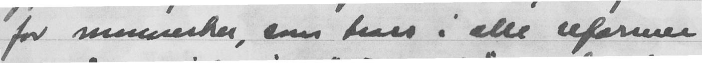for mennesker, som tross i alle ueformer
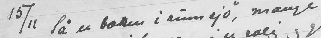15/11 Så er boken i sunn sjø, mange
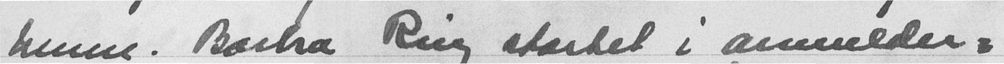henne. Barbra Ring startet i anmelder-
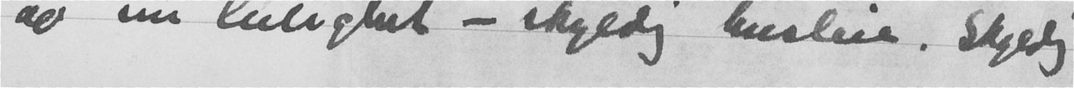av sin leilighet - skyldig husleie, skyldig
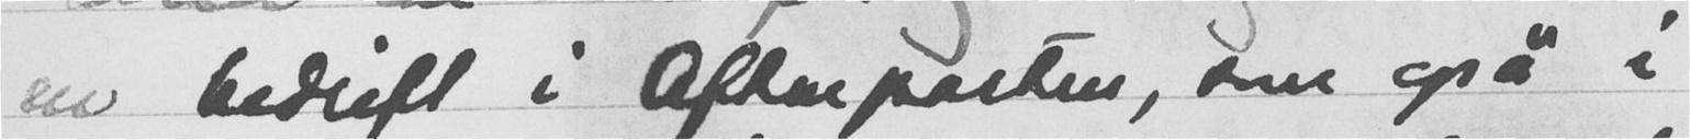en bedrift i Aftenposten, som også i
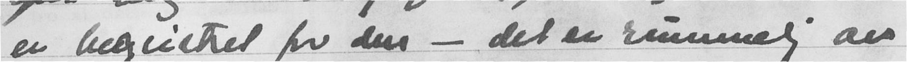er begeistret for den - det er rummelig av
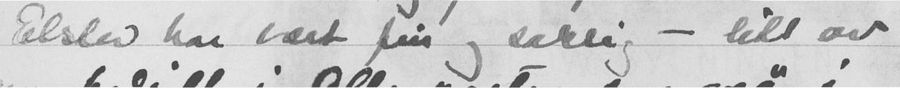Elster har vært fin og saklig - litt av
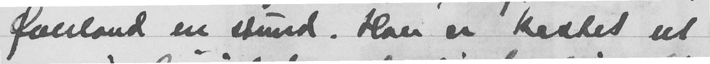Øverland en stund. Han er kastet ut
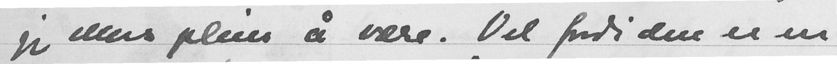jeg ellers pleier å være. Vel fordi den er en
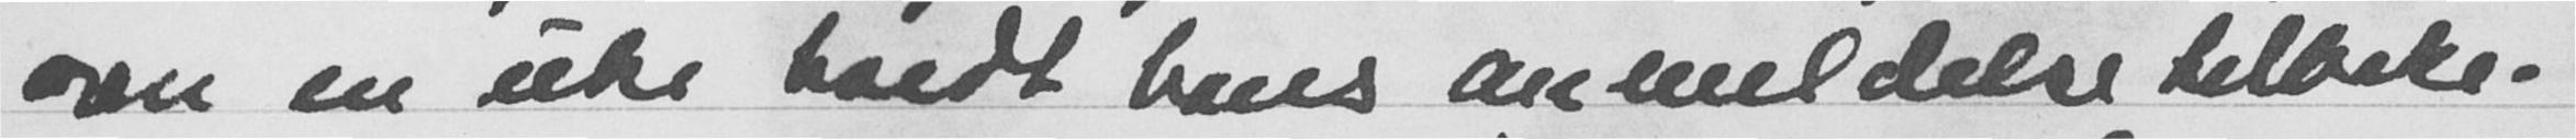over en uke holdt hans anmeldelse tilbake.
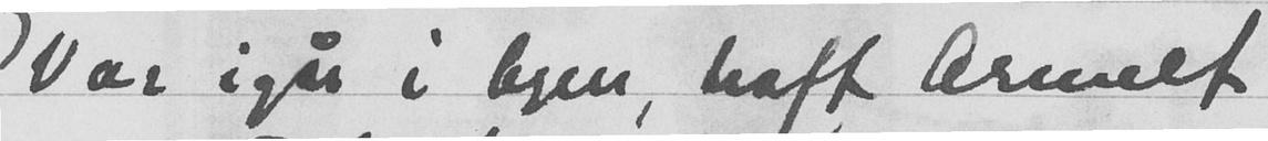Var igår i byen, traff Arnulf
